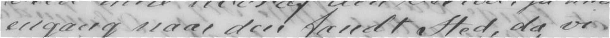engang naar den fandt Sted, da vi
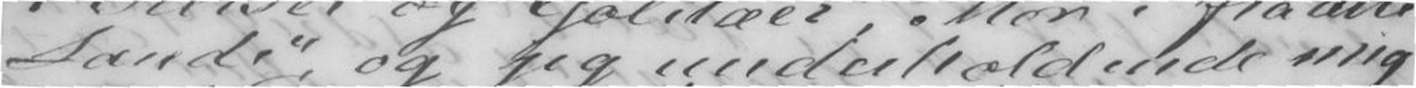Lande", og jeg underholdende mig
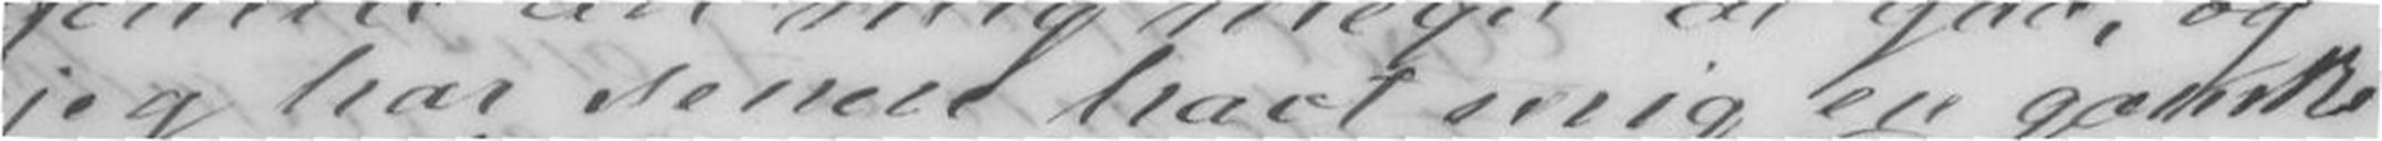jeg har senere havt mig en ganske
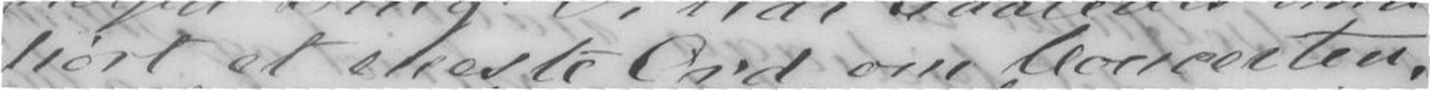hørt et eneste Ord om Concerten,
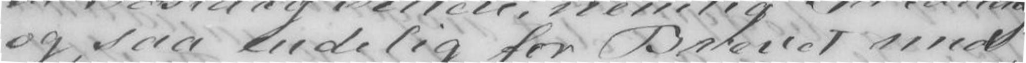og saa endelig for Brevet med
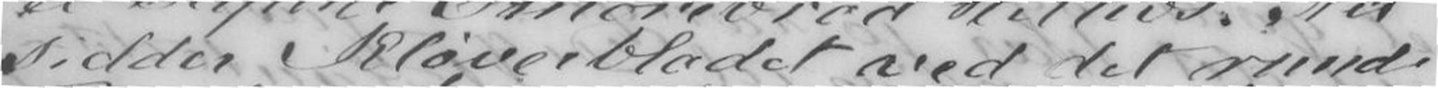sidder Kløverbladet ved det runde
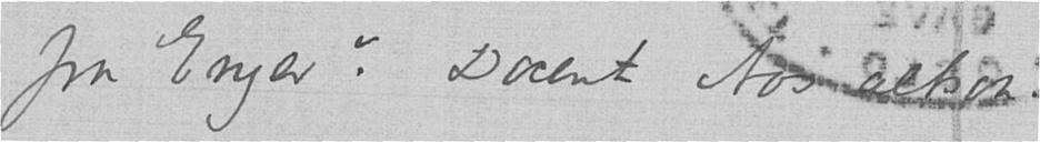fra Enger? Docent Aas altsaa.
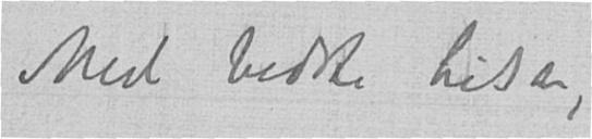Med bedste hilsen,
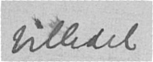billedet
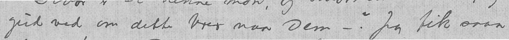gud ved om dette brev naar Dem – ? Jeg fik saan
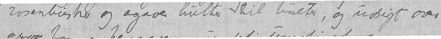rosenbusker og agaver hulter til bulter, og udsigt over
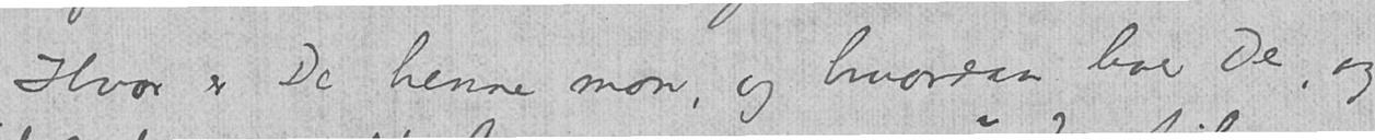Hvor er De henne mon, og hvordan lever De, og
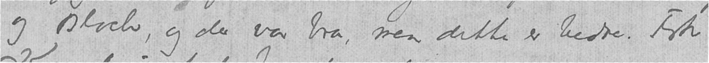og Bloch, og der var bra, men dette er bedre. Frk
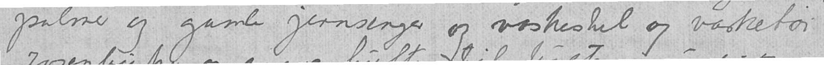palmer og gamle jernsenger og vaskestel og vasketøi
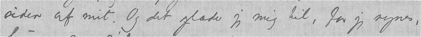siden af mit. Og det glæder jeg mig til, for jeg synes,
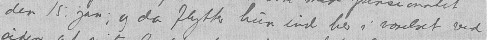den 15. jan., og da flytter hun ind her i værelset ved
